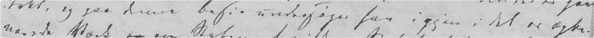tabt, og paa denne Basis undersøger han igjen i det os opbe-
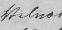Velvære
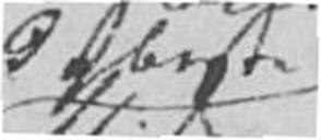bogt
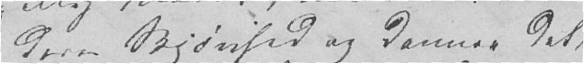deres Skjønhed og danner det
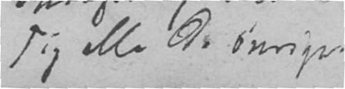sig alle de øvrige.
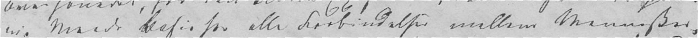vis Maade Basis for alle Forbindelser mellem Mennesker,
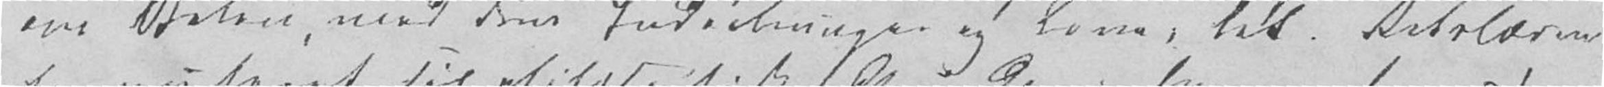om Staten, med dens Indretninger og Love, let. Retslæren
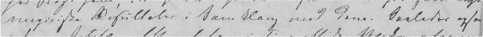empiriske Resultater i Samklang med dem. Saaledes ogsaa
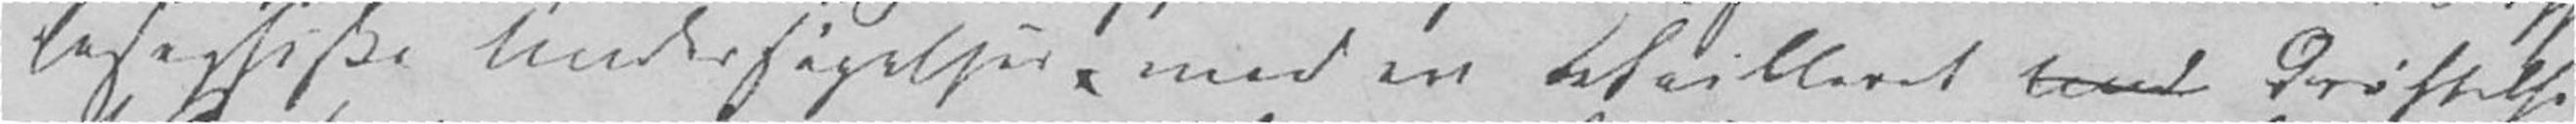losophiske Undersøgelser, med en detailleret Und Drøftelse
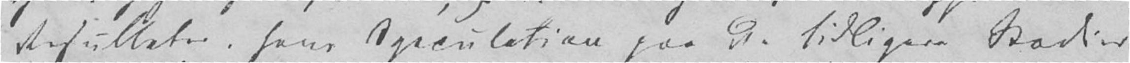Resultater, som Speculation paa de tidligere Stadier
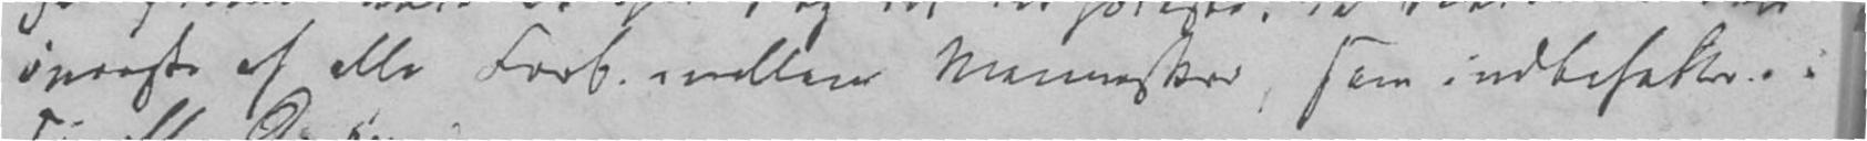øverste af alle Forb. imellem Mennesker, som indbefatter i
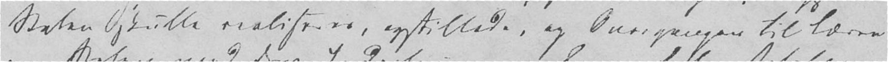Staten skulle realiseres, opstillede, og Overgangen til Læren
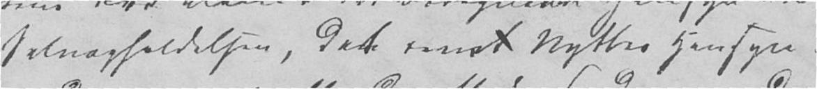Selvopholdelsen, det renet Nyttes Hensyn.
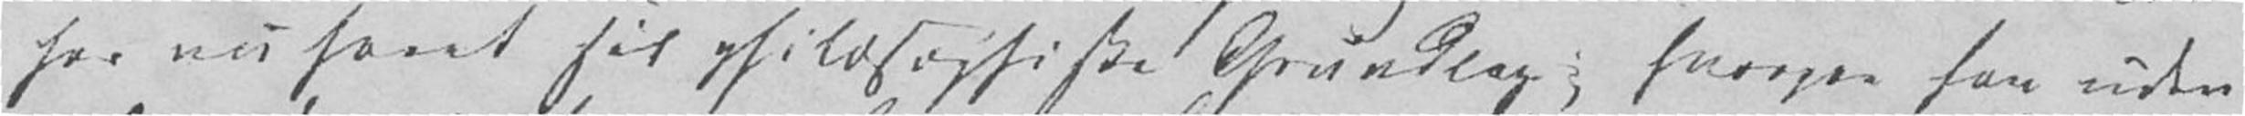har nu faaet sit philosophiske Grundlag; hvorpaa han uden
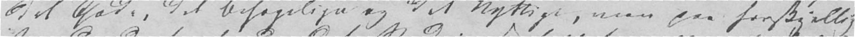det Gode, det Behagelige og det Nyttige, men paa forskjellig
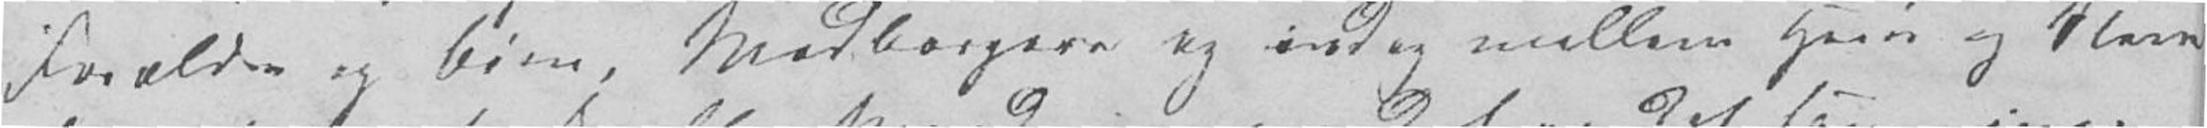Forældre og Børn, Medborgere og endog mellem Herre og Slave
